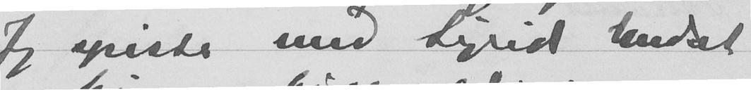Jeg spiste med Sigrid Undset
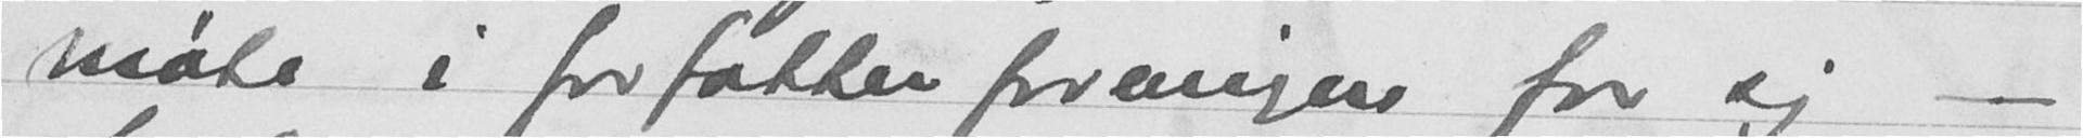møte i forfatterforeningen for sig -
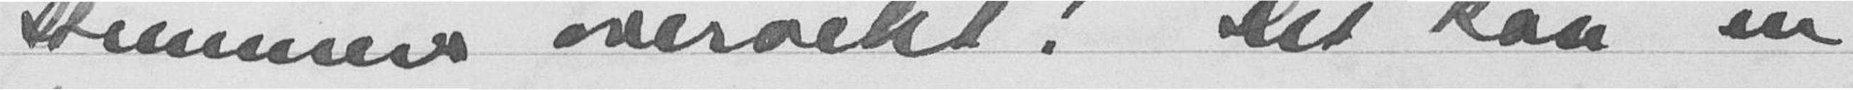stemmers overvekt! Det kan en
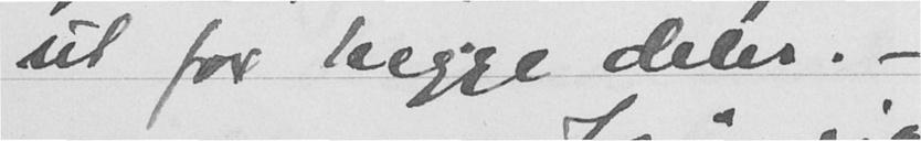ut for begge deler. -
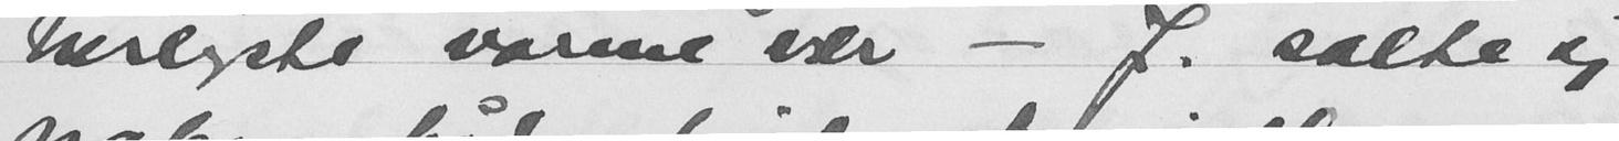herligste varme vær - J. satte sig
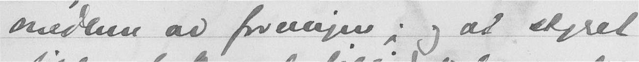medlem av foreningen; og at styret
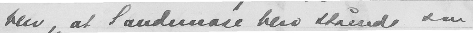blev, at Sandemose blir stående, som
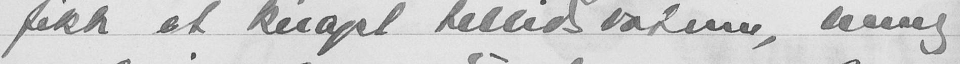fikk et knapt tillidsvotum, nemlig
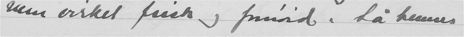men virket frisk og fornøyd. Så hennes
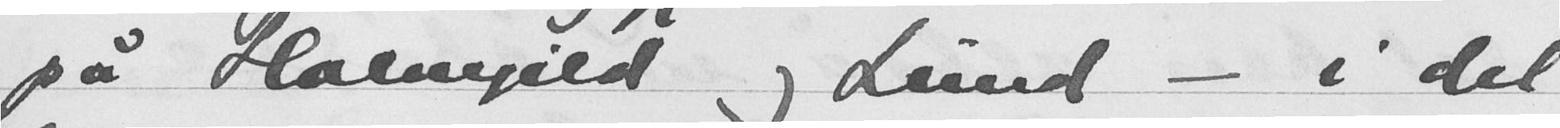pa Holmgild og Lund - i det
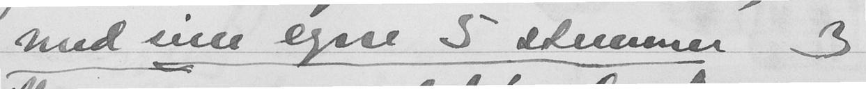med sine egne 5 stemmer 3
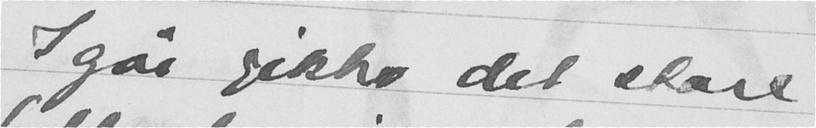Igår gikk det store
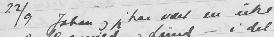23/9 Johan og jeg har vært en uke
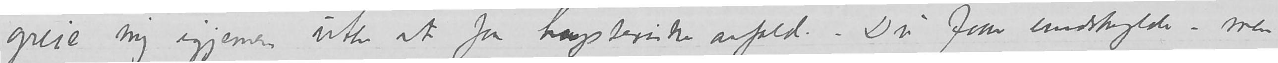greie mig igjennem uten at faa hysteriske anfald. Du faar undskylde – men
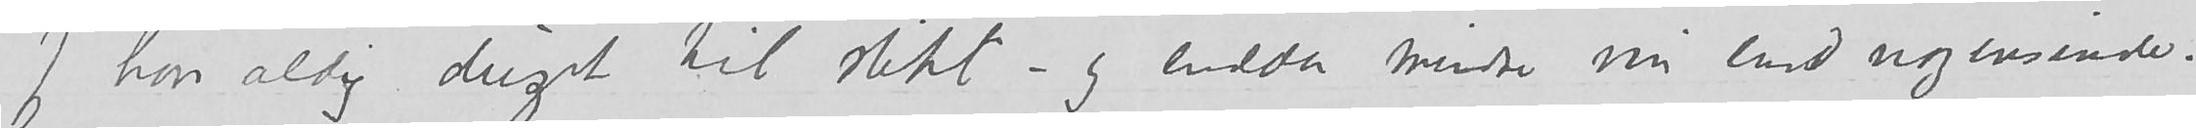Jeg har aldrig duget til slikt – og endda mindre nu end nogensinde.
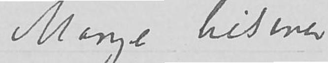Mange hilsener
